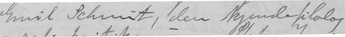Emil Schmit, den kjende filolog
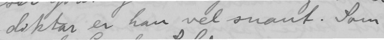diktar er han vel snaut. Som
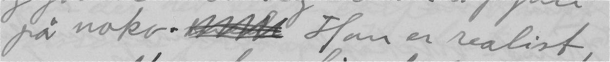på noko.  Han er realist,
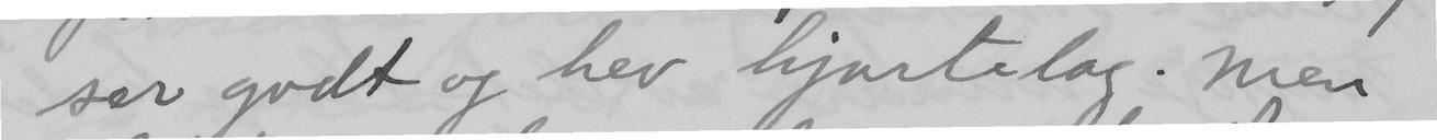ser godt og hev hjartelag. Men
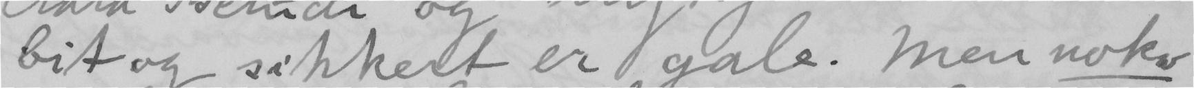bit og sikkert er gale. Men noko
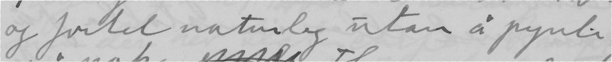og fortel naturleg utan å pynte
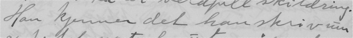Han kjenner det han skriv um
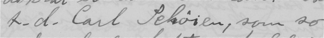t.d. Carl Schöien, som so
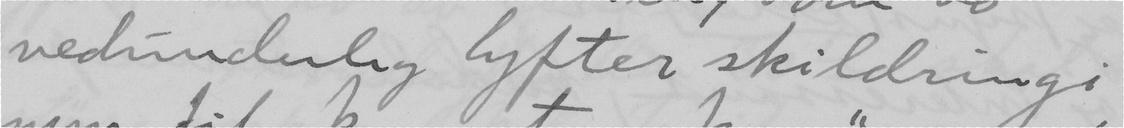vedunderleg lyfter skildringi
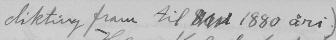dikting fram til  1880 åri.
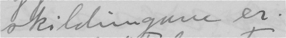skildringane er.
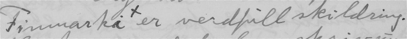Finmarki er verdfull skildring.
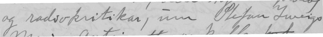og radiokritikar, um Stefan Zweigs
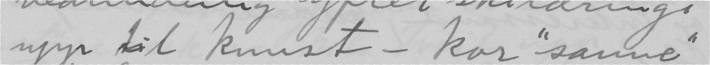upp til kunst – kor "sanne"
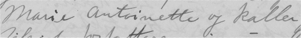Marie Antoinette og kaller
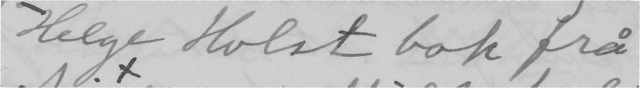Helge Holst bok frå
